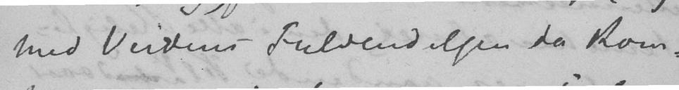med Verdens Fuldendelsen da kom-
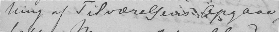ning af Tilværelsens Opgave
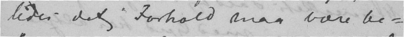ledes det Forhold maa være be-
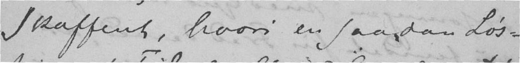skaffent, hvori en saadan Løs-
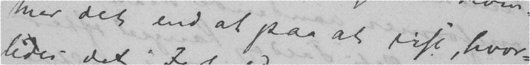mer det end at paa at vise, hvor-
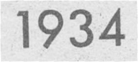﻿1934
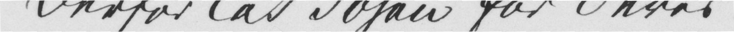Derfor Tak Ibsen for Deres
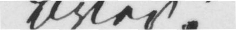Brev!
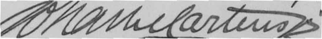Johan Martens F.t. formand
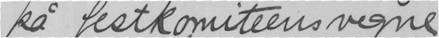på festkomiteens vegne
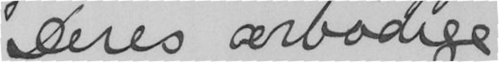Deres ærbødige
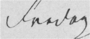Fredag
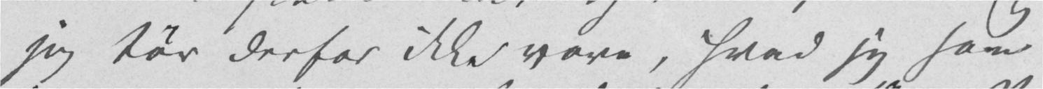jeg tør derfor ikke vove, hvad jeg saa
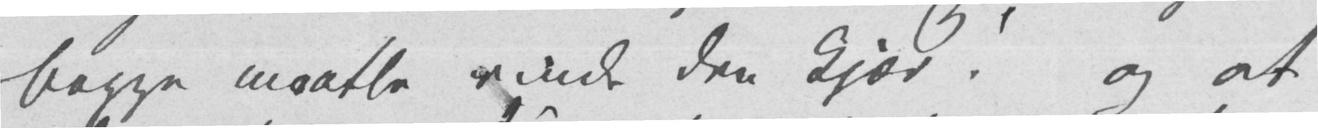begge maatte vinde den kjær! og at
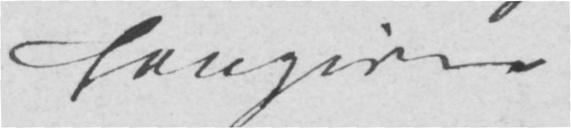hengivne
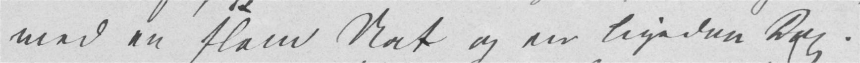med en slem Nat og en ligedan Dag.
